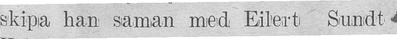skipa han saman med Eilert sundt
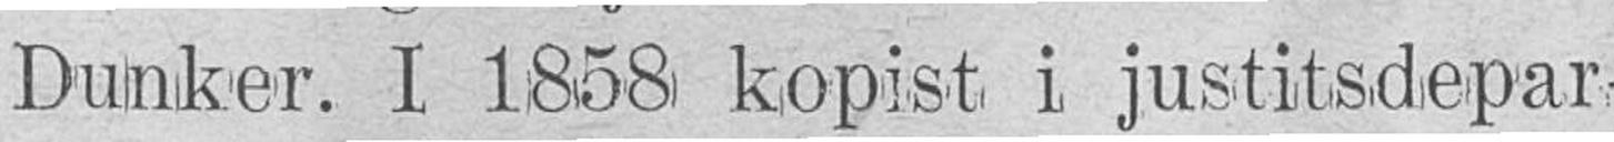Dunker. I 1858 kopist i justitsdepar-
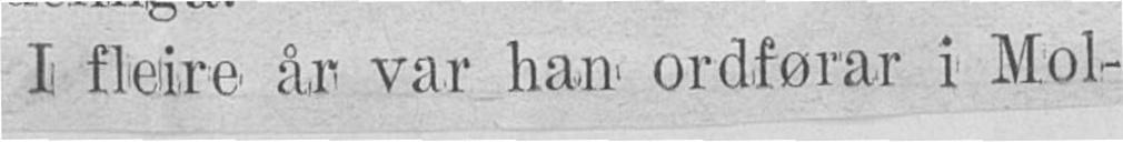I fleire år var han ordførar i Mol-
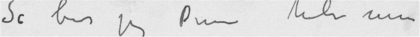Så ber jeg Dem hilse min
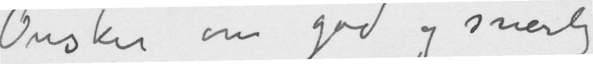Ønsker om god og snarlig
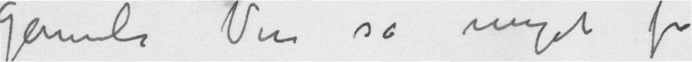gamle Ven så meget fra
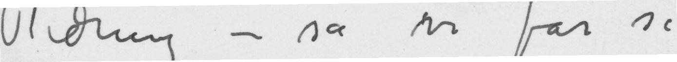Bedring – så vi får se
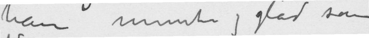ham munter og glad som
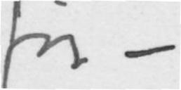før –
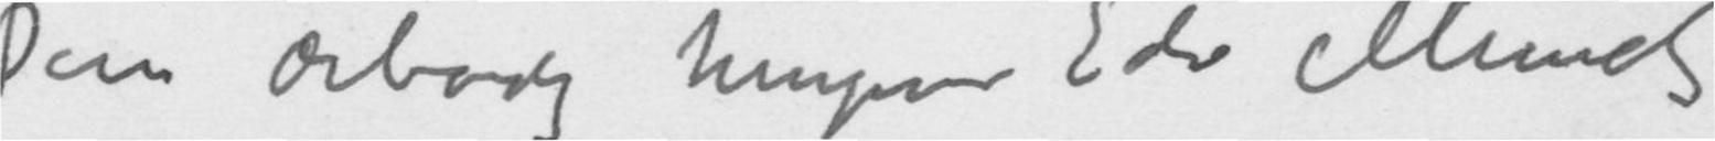Deres ærbødig hengivne Edv Munch
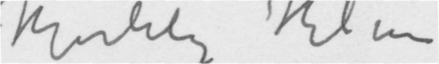Hjertelig Hilsen
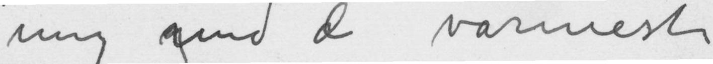mig ogmed de varmeste
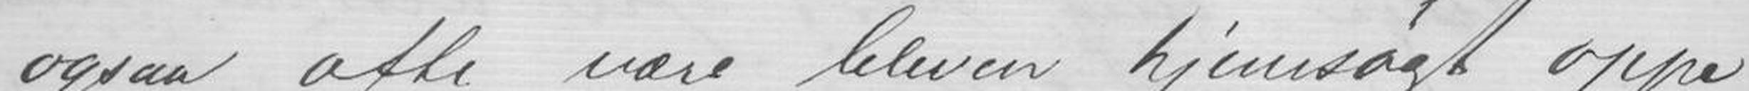ogsaa ofte være bleven hjemsøgt oppe
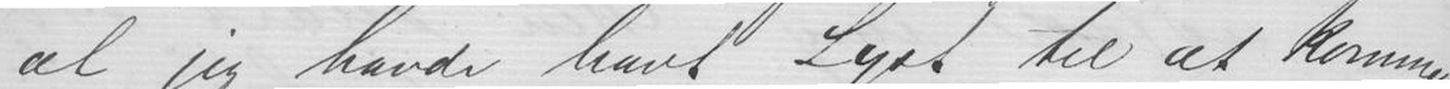at jeg havde havt Lyst til at komme
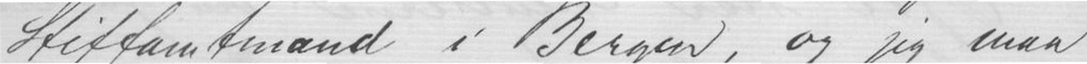Stiftamtmand i Bergen, og jeg maa
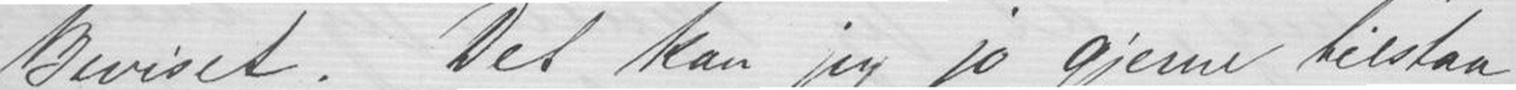Beviset. Det kan jeg jo gjerne tilstaa,
